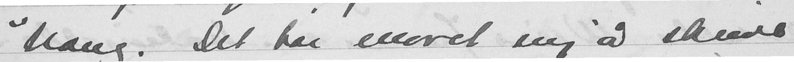haug. Det har moret mig å skrive
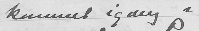kommet igang i
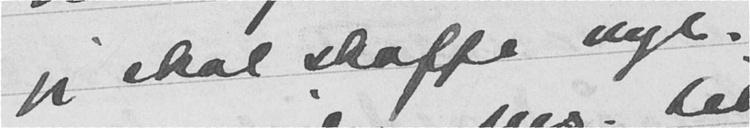vi skal skaffe nye.
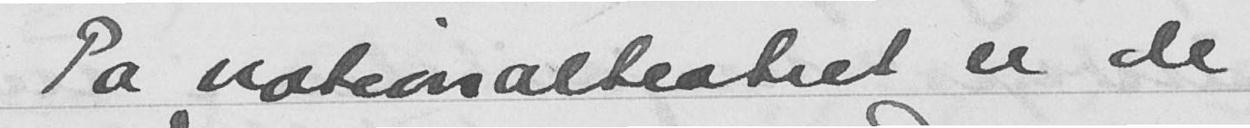På nationaltheatret er de
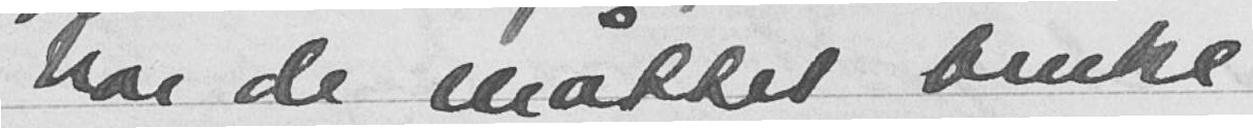har de måttet bruke
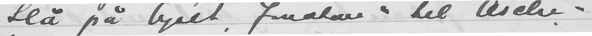"Slå på lyset, Jonatan" til Asche-
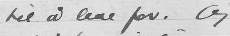til å leve for. Og
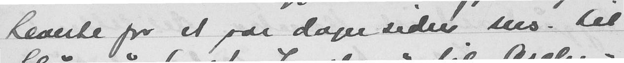Leverte for et par dage siden ms. til
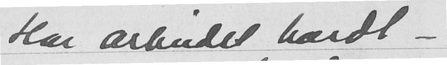Har arbeidet hardt -
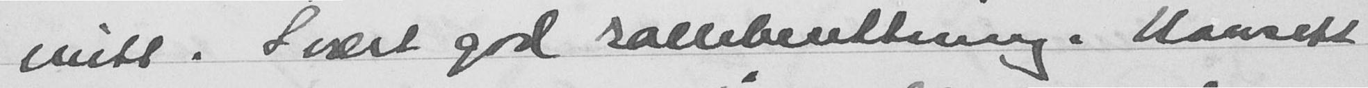mitt. Svært god rollebesettning. Uansett
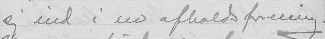sig ind i en afholdsforening.
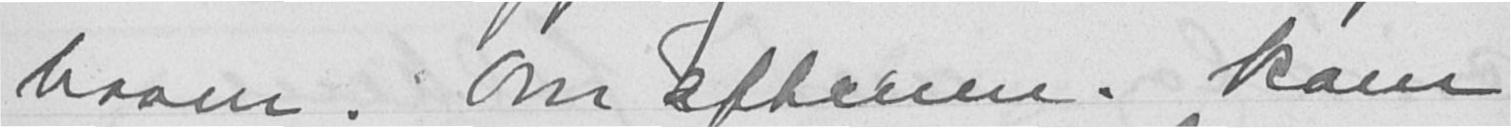haven. Om efterm. kom
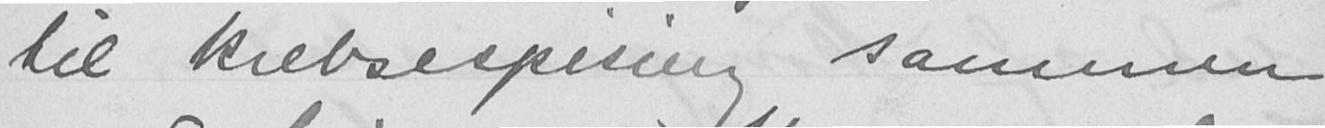til krebsespising sammen
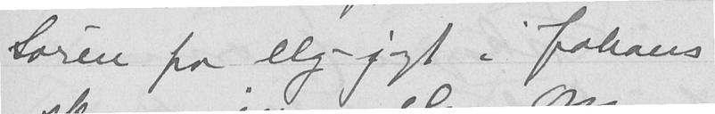Søren fra elg-jagt i Johans
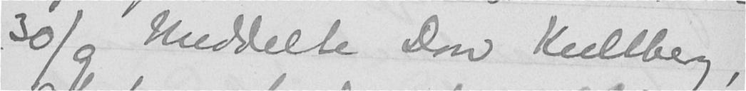30/9 Meddelte Don Kullberg,
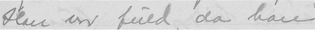Han var fuld, da han
